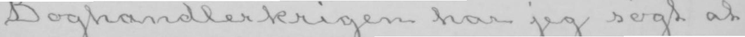Boghandlerkrigen har jeg søgt at
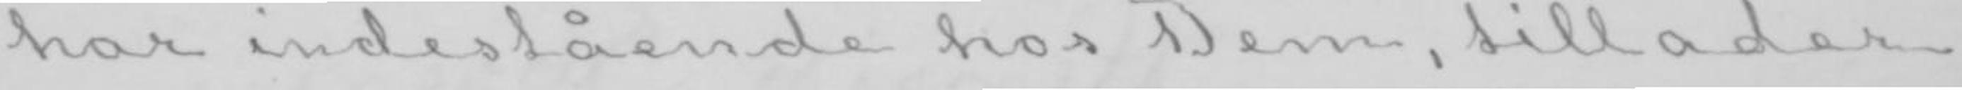har indestående hos Dem, tillader
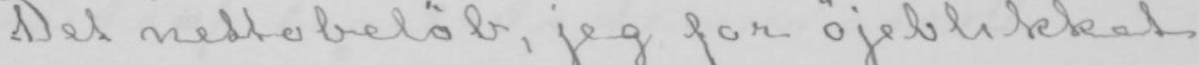Det nettobeløb, jeg for øjeblikket
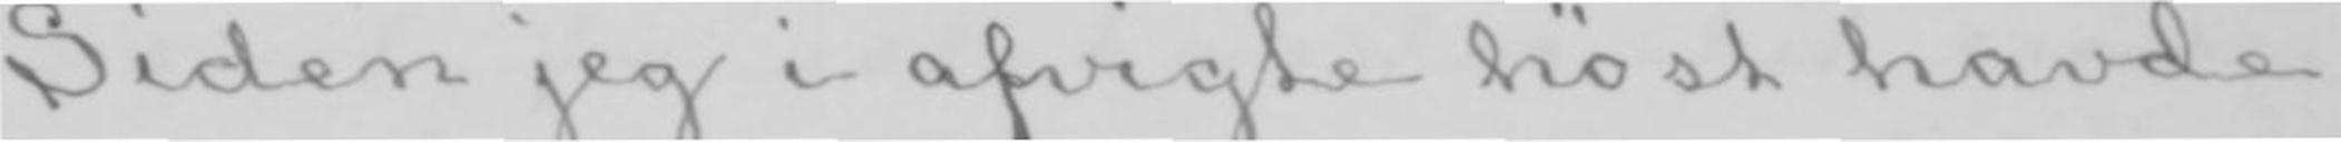Siden jeg i afvigte høst havde
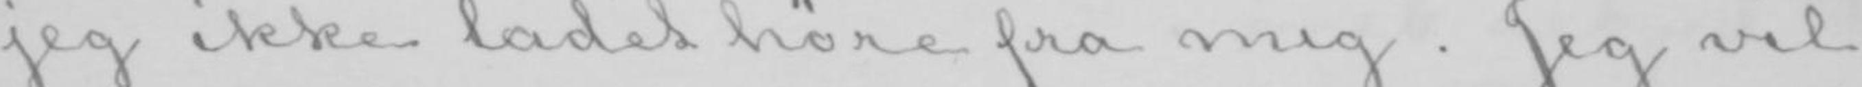jeg ikke ladet høre fra mig. Jeg vil
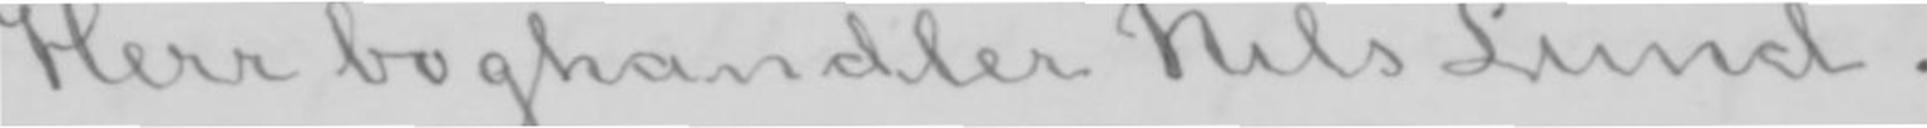Herr boghandler Nils Lund.
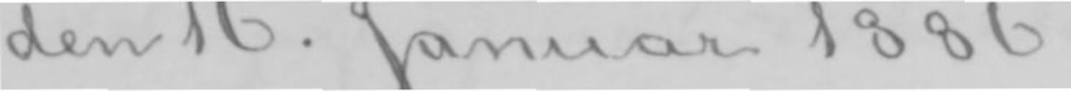den 16. Januar 1886.
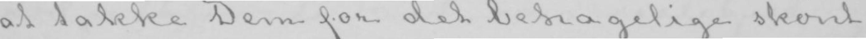at takke Dem for det behagelige skønt
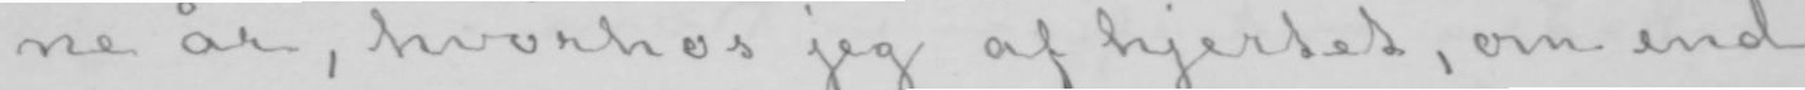ne år, hvorhos jeg af hjertet, om end
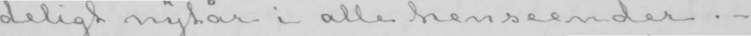deligt nytår i alle henseender.-
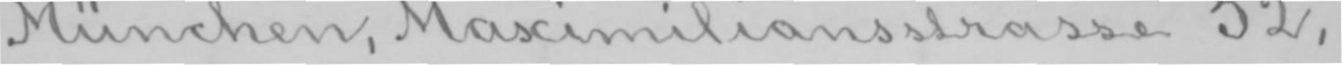München, Maximilianstrasse 32,
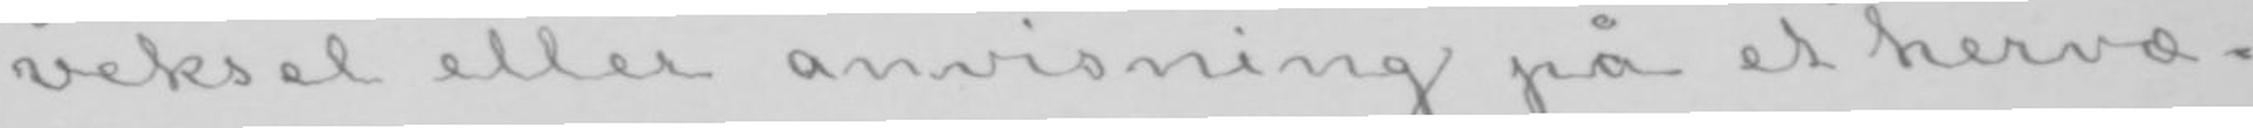veksel eller anvisning på et hervæ-
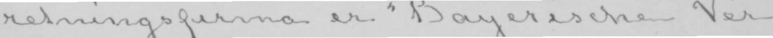retningsfirma er «Bayerische Ver-
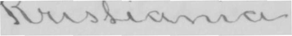Kristiania.
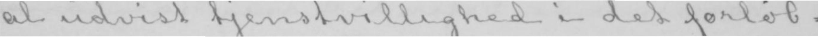al udvist tjenstvillighed i det forløb-
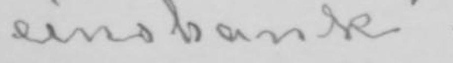einsbank».
